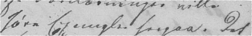høre Exempler herpaa. Det
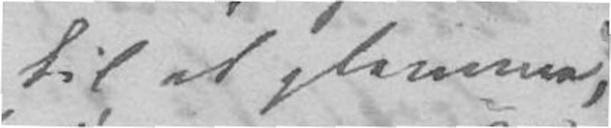til at glemme,
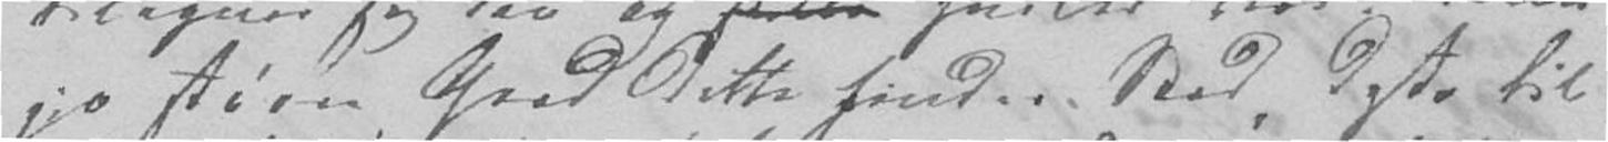jo større Grad dette finder Sted, desto til-
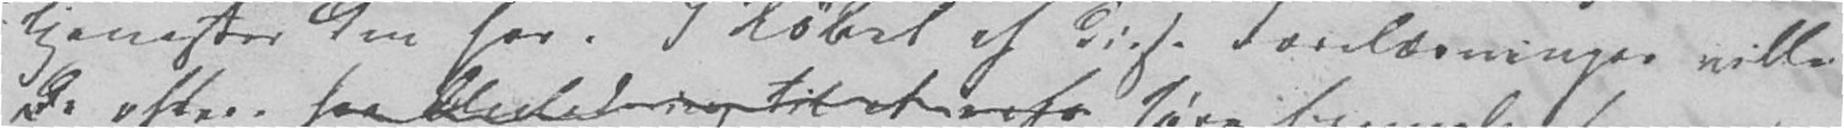rtjenester den har. I Løbet af disse Forelæsninger ville
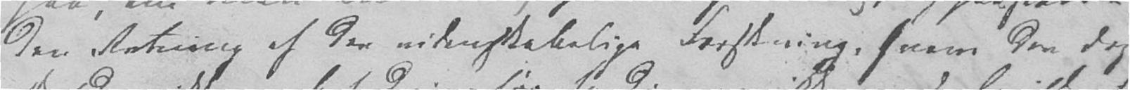den Retning af den videnskabelige Forskning, hvem den dog
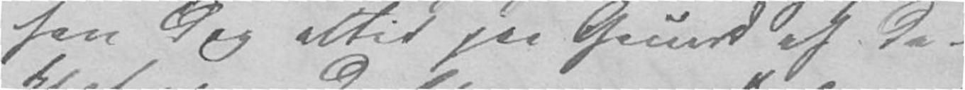han dog altid paa Grund af de
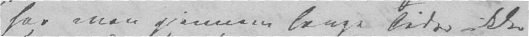har man gjennem lange Tider ikke
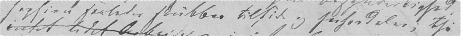sophien saaledes skubbes tilside og forfordeles; thi
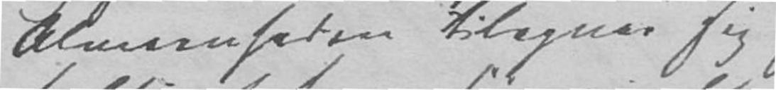Almeenheden tilegner sig
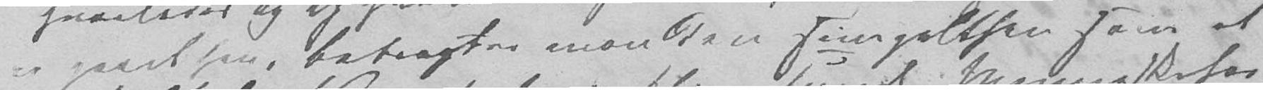er gaaet hen, betragter man den simpelthen som et
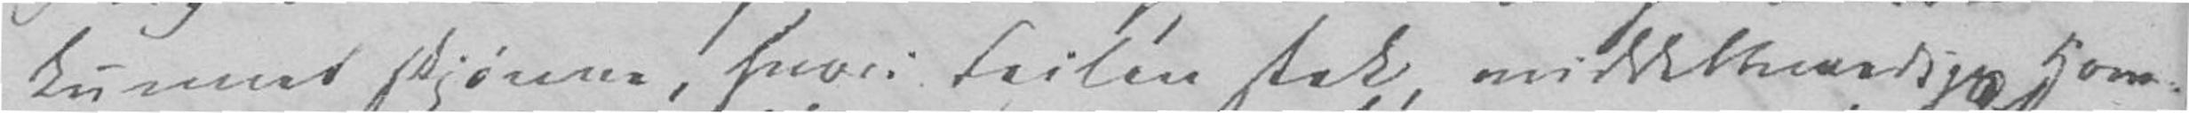kunnet skjønne, hvori Feilen stak, middelmaadige Hove-
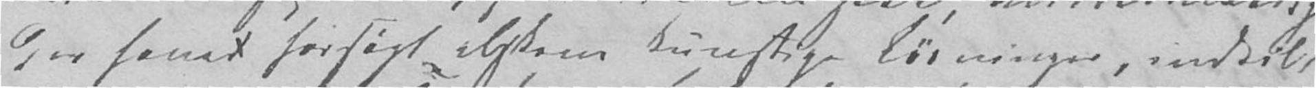der havet forsøgt alskens kunstige Løsninger, indtil
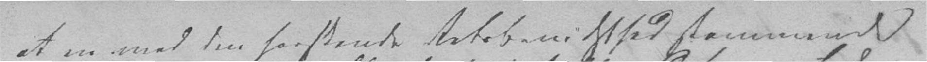at en med den herskende Retsbevidsthed stemmende
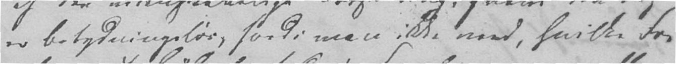er betydningsløs, fordi man ikke veed, hvilke Fo-
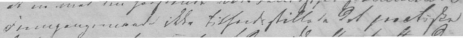Fremgangsmaade ikke tilfredsstillede det practiske
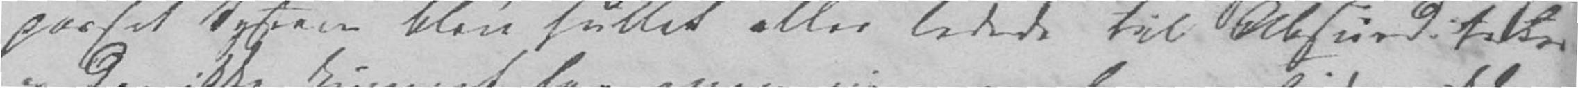passet System blev tullet eller ledede til Absurditeter
